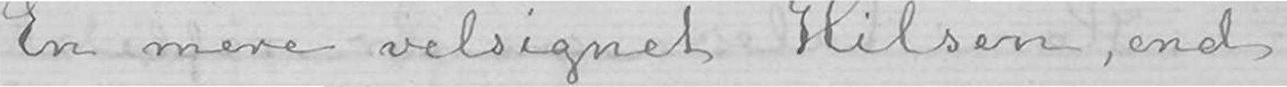En mere velsignet Hilsen, end
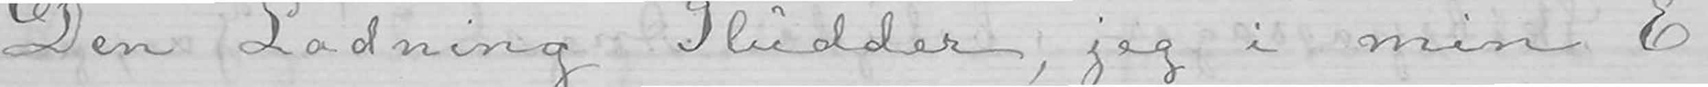Den Ladning Sludder, jeg i min E-
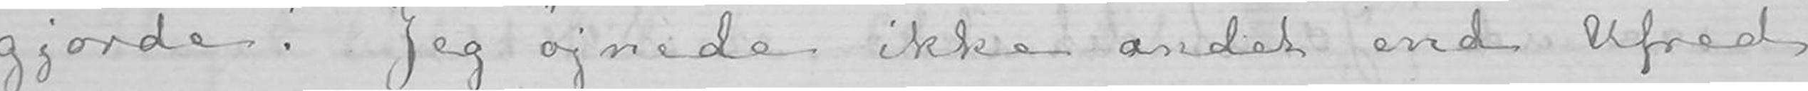gjorde! Jeg øjnede ikke andet end Ufred
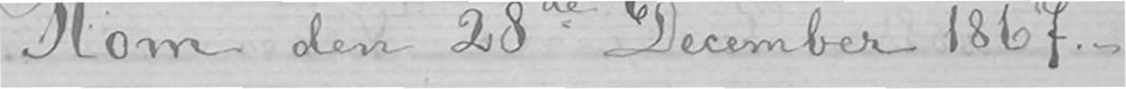Rom den 28de December 1867.-
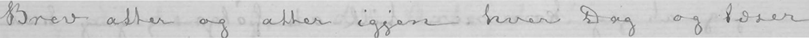Brev atter og atter igjen hver Dag og læser
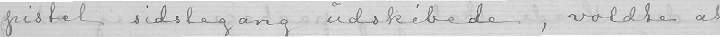pistel sidstegang udskibede, voldte at
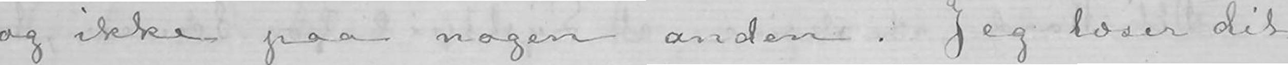og ikke paa nogen anden. Jeg læser dit
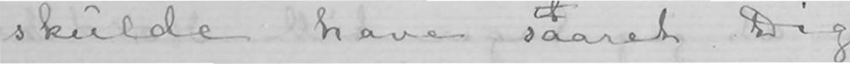skulde have saaret Dig.-
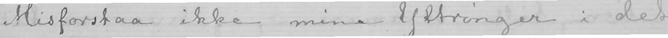Misforstaa ikke mine Yttringer i det
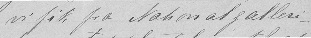vi fik fra Nationalgalleri-
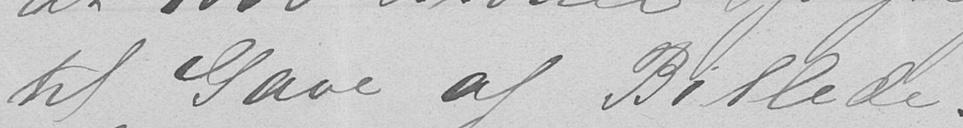til Gave af Billede.
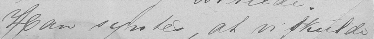Han syntes, at vi skulde
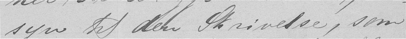syn til den Skrivelse, som
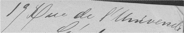19 Rue de l'Universite
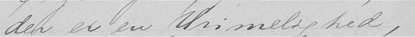der er en Urimelighed
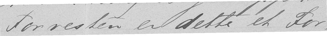Forresten er dette et For-
